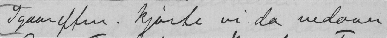I gaar eften. kjørte vi da nedover
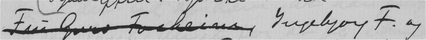, Ingebjørg F. og
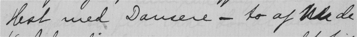Hest med Dansere - to af  de
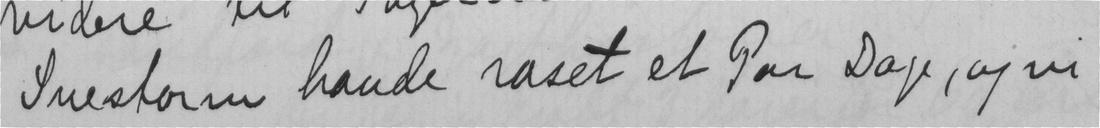Snestorm havde raset et Par Dage, og vi
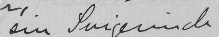sin Svigerinde
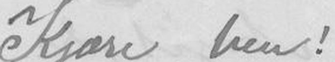Kjære Ven!
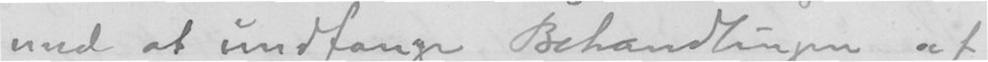med at undfange Behandlingen af
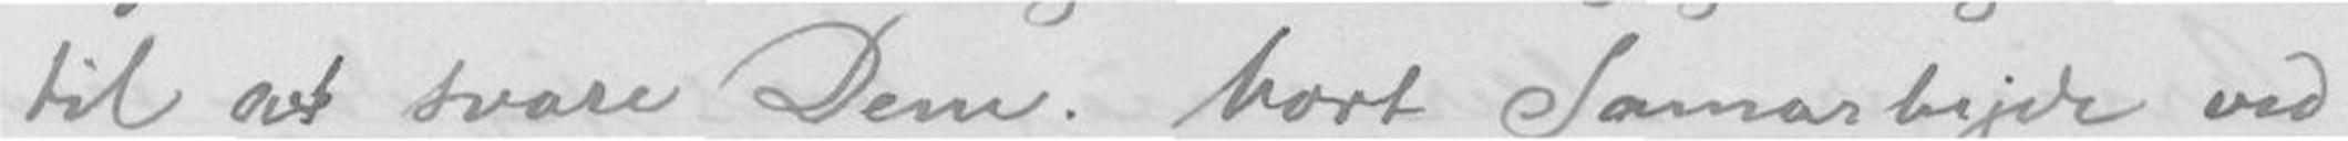til at svare Dem. Vort Samarbejde ved
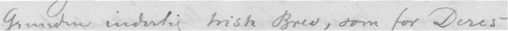Grunden inderlig triste Brev, som for Deres -
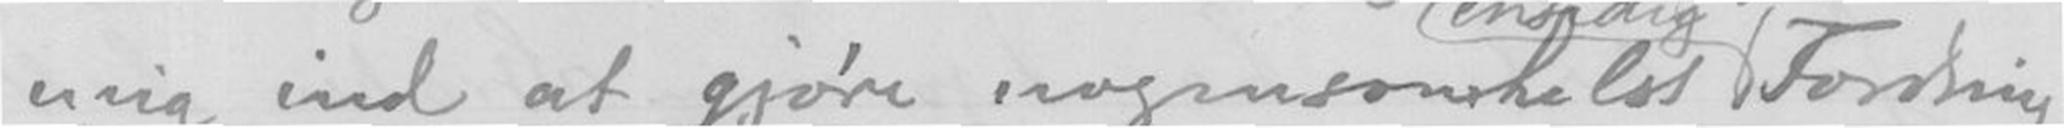mig ind at gjøre nogensomhelst Fordring
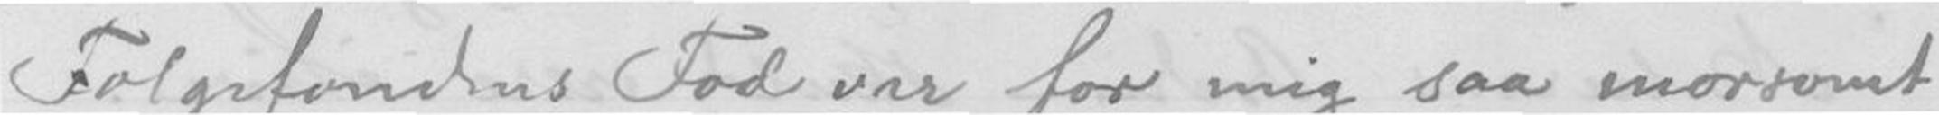Folgefonden Fod var for mig saa morsomt
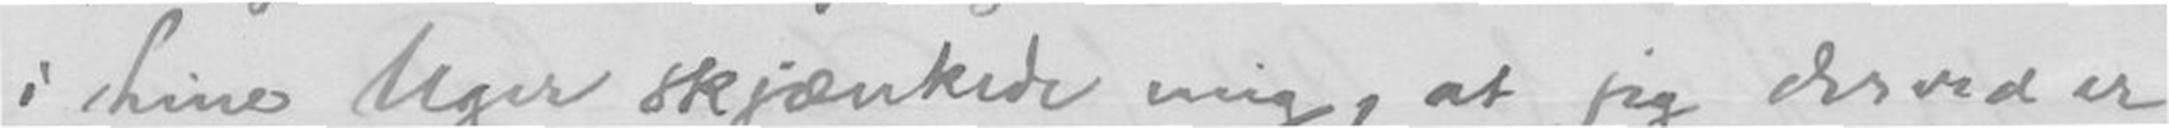i hine Uger skjænkede mig, at jeg der ved er
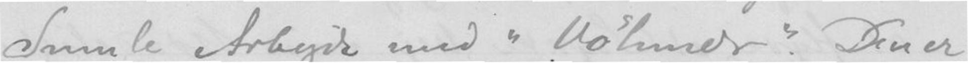Smule Arbejde med Vöhmede. Den er
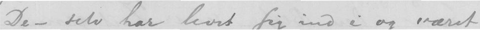De - selv har levet sig ind i og været
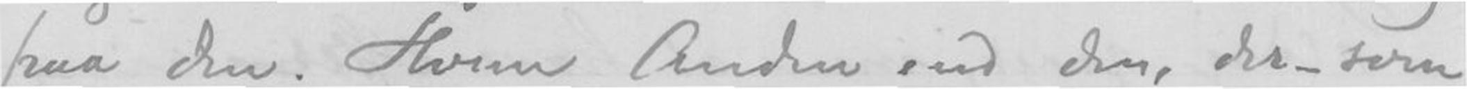paa Den. Hvem Anden end den, der - som
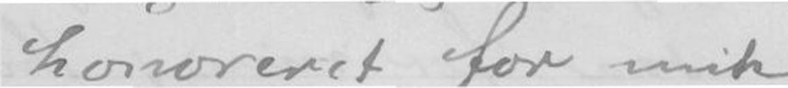honoreret for mit
